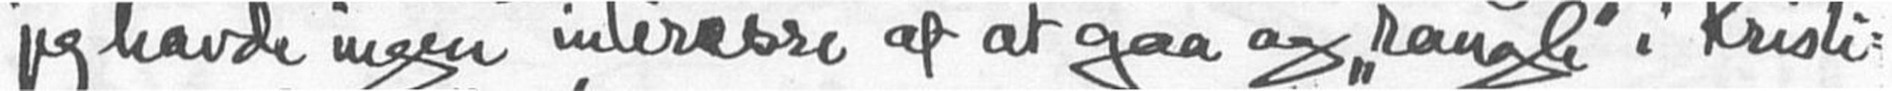jeg havde ingen interesse af at gaa og "rangle" i Kristi-
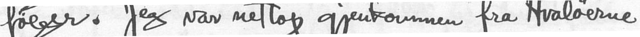følger. Jeg var nettop hjemkommen fra Hvaløerne
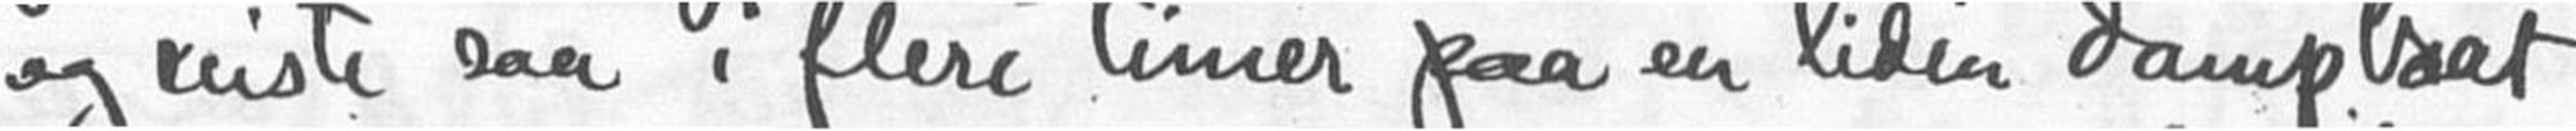og reiste saa i flere timer paa en liden dampbaad
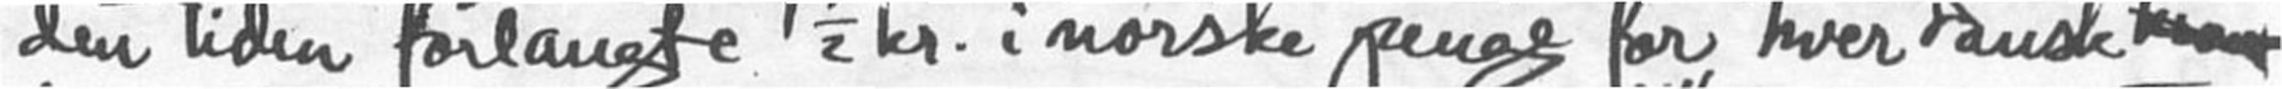den tiden forlangte 1 1/2 kr. i norske penge for hver dansk
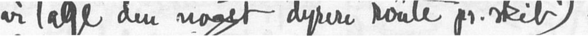vi tage den noget dyrere route pr. skib).
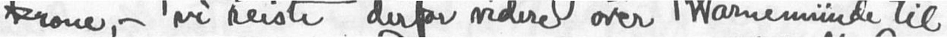krone,- vi reiste derfor videre over Warnemunde til
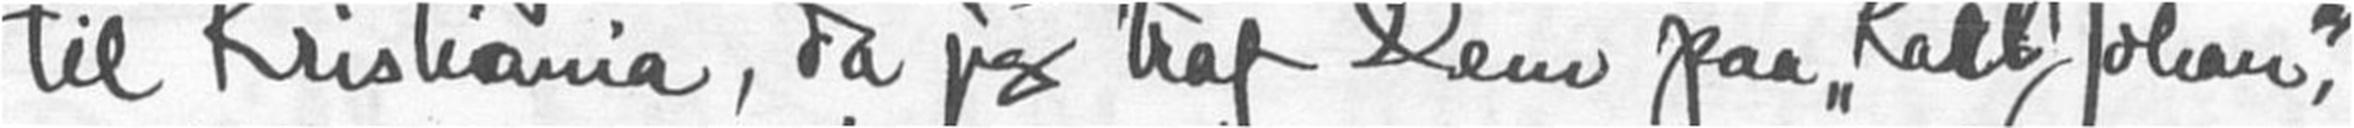til Kristiania, da jeg traf Dem paa "Karl Johan",-
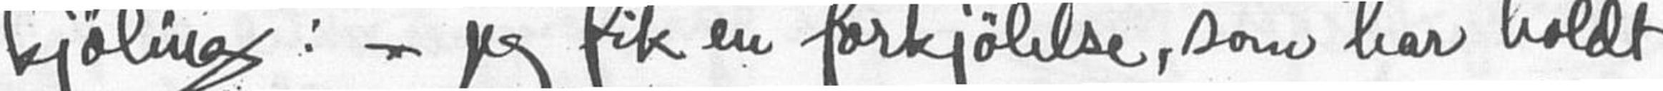kjøling : - jeg fik en forkjølelse, som har holdt
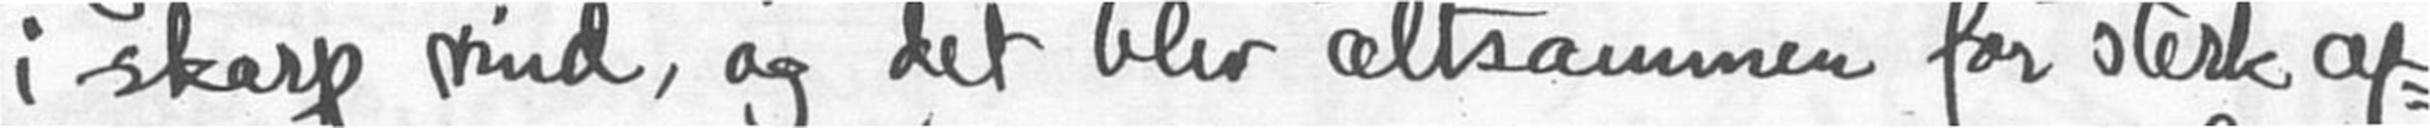i skarp vind, og det blev altsammen for sterk af-
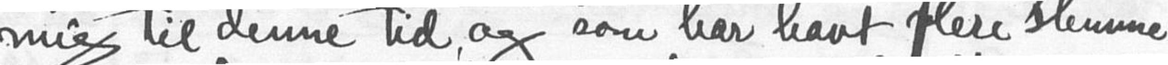mig til denne tid, og som har havt flere slemme
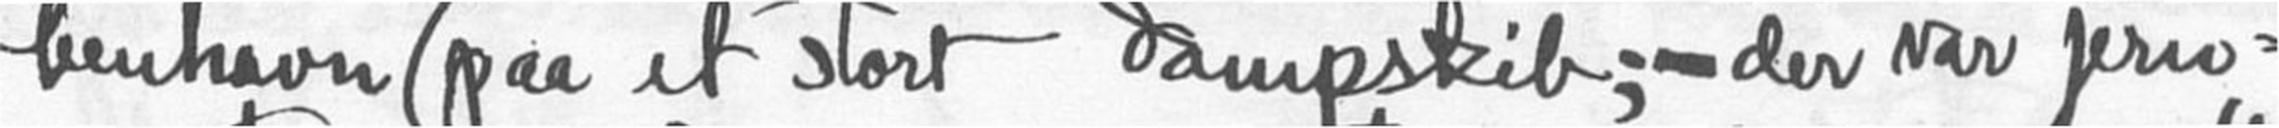benhavn (paa et stort dampskib ;- der var jern-
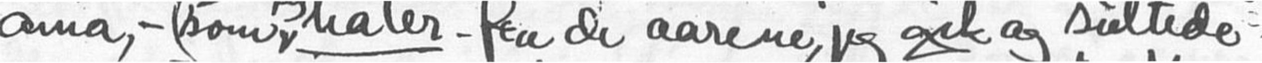ania,- (som jeg hater fra de aarene, jeg gik og sultede
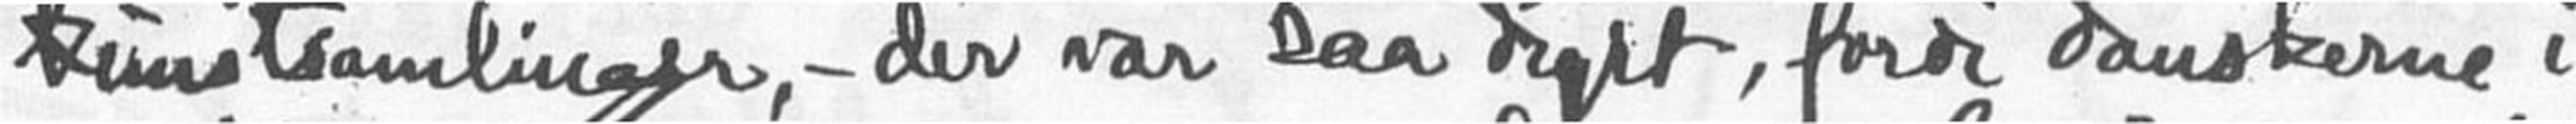kunstsamlinger, - der var saa dyrt, fordi danskerne i
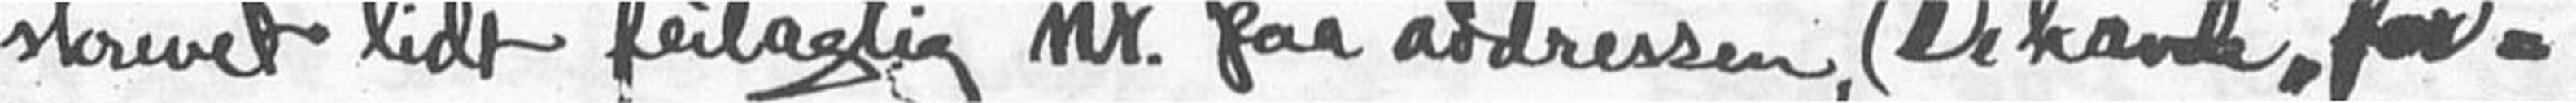skrevet lidt feilagtig nr. paa adressen, (De havde "for-
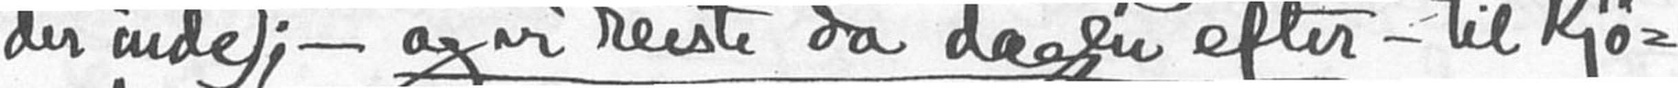der inde); -og vi reiste da dagen efter - til Kjø-
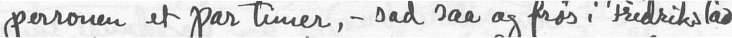perronen et par timer,- sad saa og frøs i Fredrikstad
